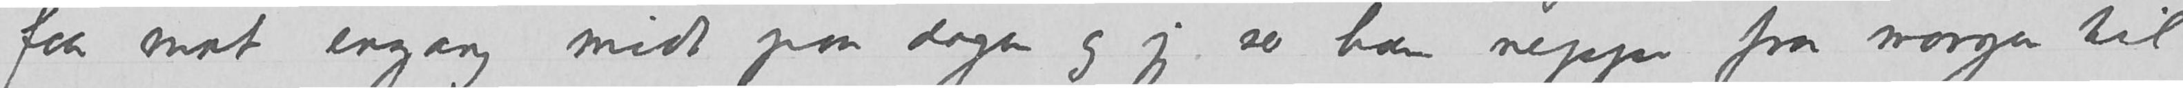faar mat engang midt paa dagen og jeg ser ham neppe fra morgen til
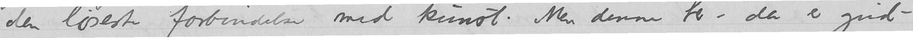den løseste forbindelse med kunst. Men denne her - den er gud-
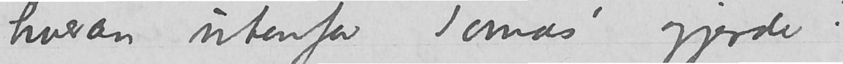hveran utenfor Tomas’ gjerde.»
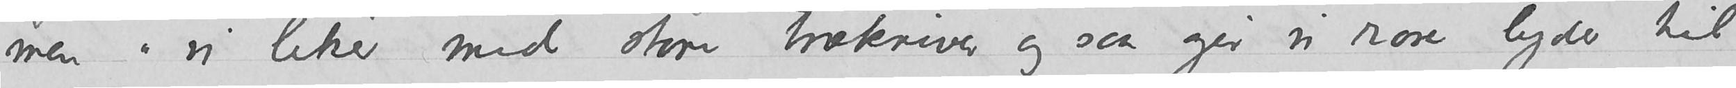men «vi leker med store trækniver og saa gir vi rare lyder til
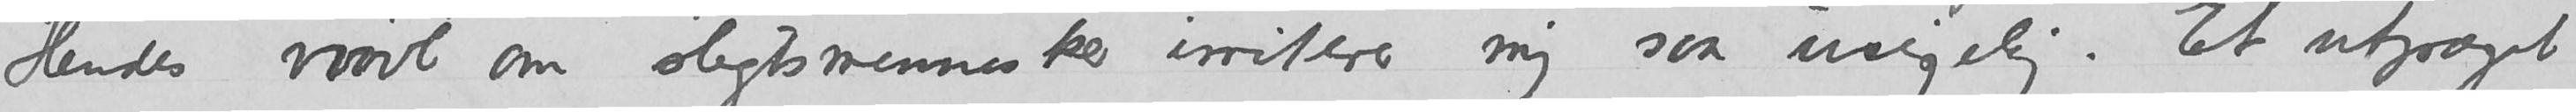Hendes vrøvl om slegtsmennesker irriterer mig saa usigelig. Et utpræget
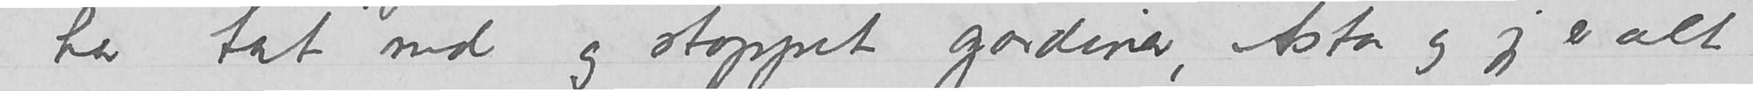Jeg har tat ned og stoppet gardiner, Asta og jeg er alt
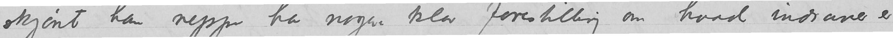skjønt han neppe har nogen klar forestilling om hvad indianer er
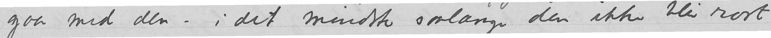gaar med den – i det mindste saalænge den ikke blir rørt
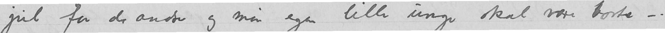jul for de andre og min egen lille unge skal være borte -.
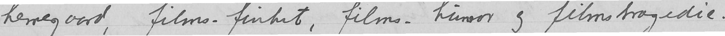herregaard, films-frihet, films-humor og filmstragedie.
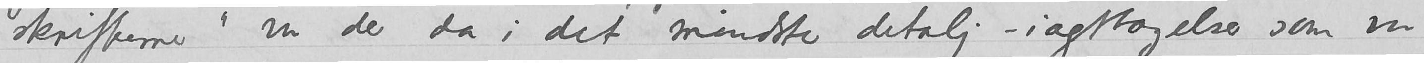skrifterne» var der da i det mindste detalj-iagttagelser som var
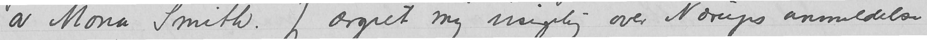av Mona Smith. Jeg ærgret mig usigelig over Nærups anmeldelse
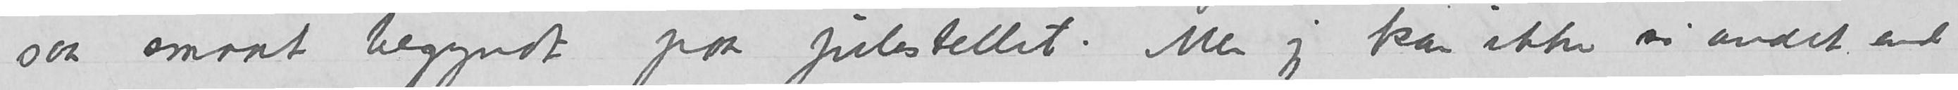saa smaat begyndt paa julestellet. Men jeg kan ikke si andet end
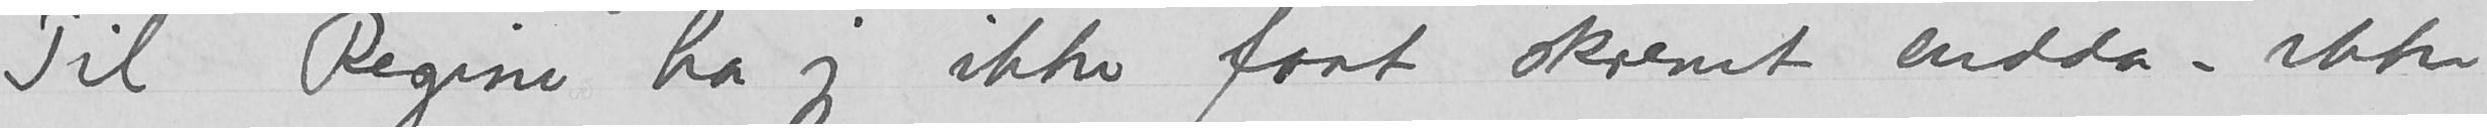Til Regine har jeg ikke faat skrevet endda – ikke
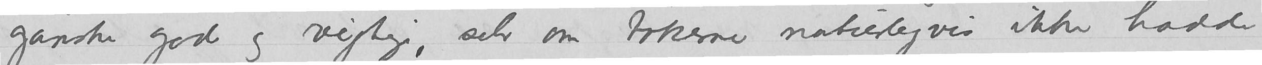ganske gode og vigtige, selv om bøkerne naturligvis ikke hadde
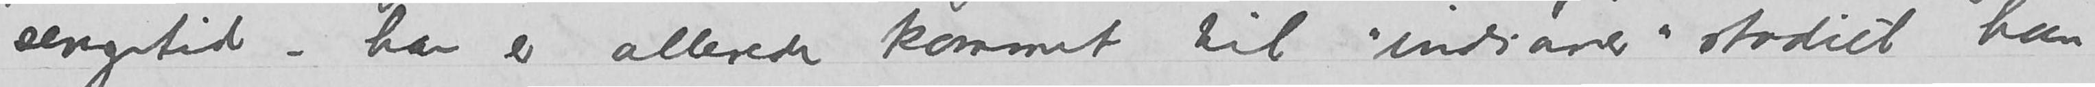sengetid – han er allerede kommet til «indianer» stadiet han
